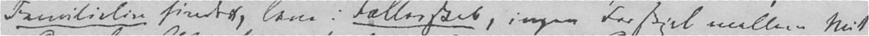Familieliv findes, leve i Fælledsskab, ingen Forskjel mellem Mit
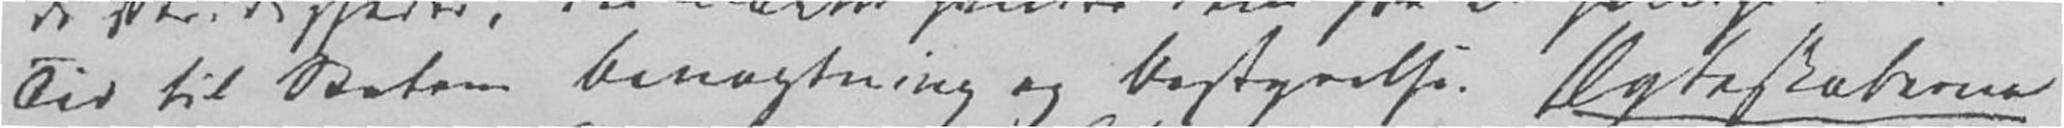Tid til Statens Bevogtning og Bestyrelse. Ægteskaberne
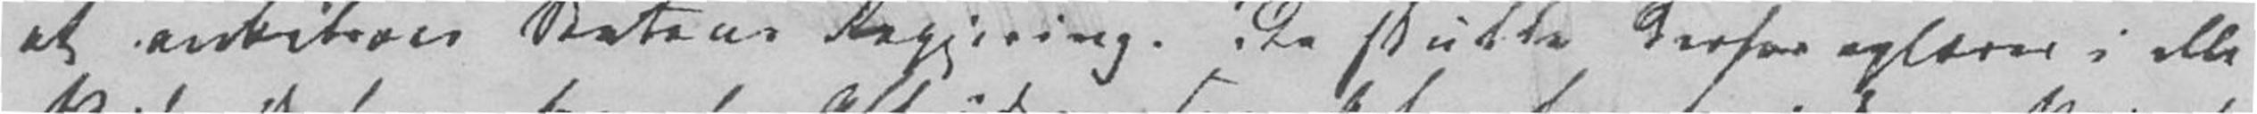at anbetroe Statens Regjering. De skulde derfor oplæres i alle
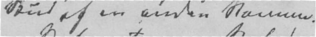Skud af en anden Stamme.
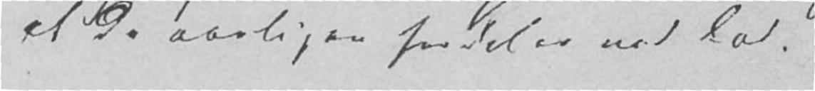at de aarligen fordeles ved Lod.
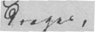drages
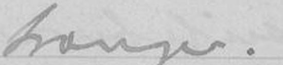trænger.
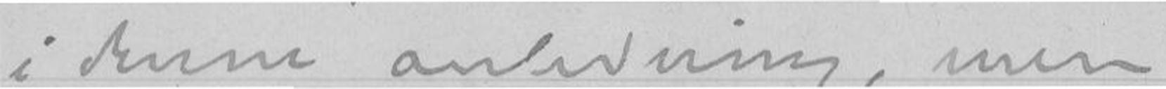i denne anledning, men
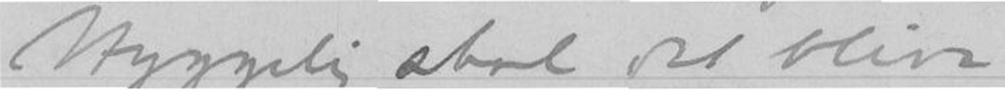Hyggelig skal det blive
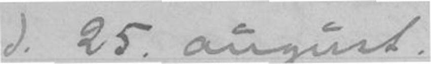d. 25. august.
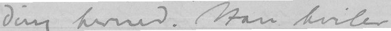ding hermed. Han hviler
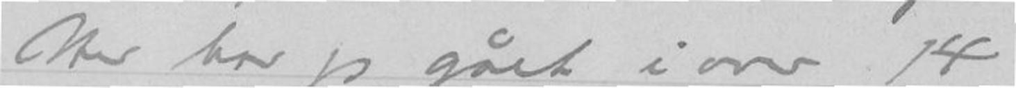Her har jeg gået i over 14
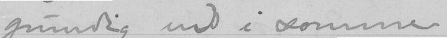grundig ud i sommer,
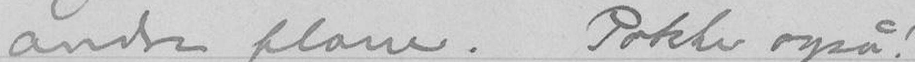andre plane. Pokker også!
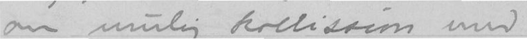om mulig kollission med
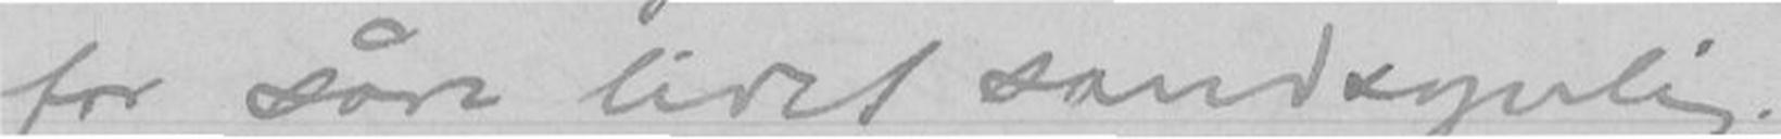for såre lidet sandsynlig.
